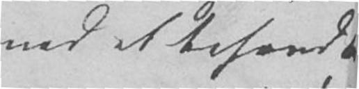ved at behandle
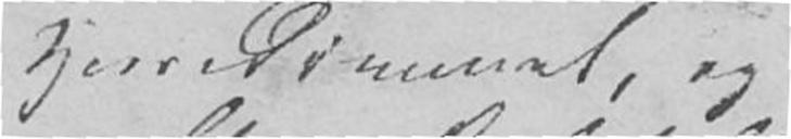Herredømmet, og
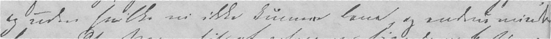og uden hvilke vi ikke kunne leve, og endnu mindre
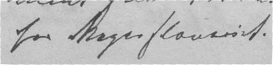for Negerslaveriet.
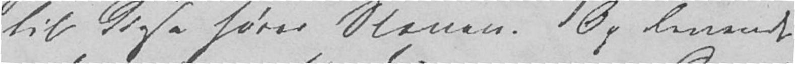til disse hører Slaven. Og levende
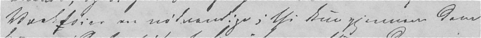Værktøier ere nødvendige; thi kun gjennem dem
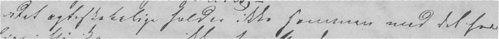Det ægteskabelige falder ikke sammen med det her-
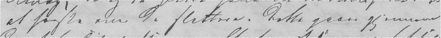at herske over de slettere. Dette gaaer gjennem
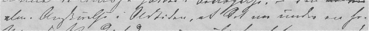alm. Anskuelse i Oldtiden, at det var under en fri
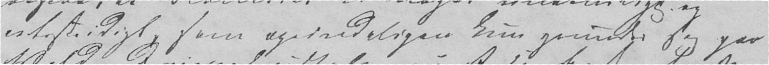retstridigt, som oprindeligen kun grunder sig paa
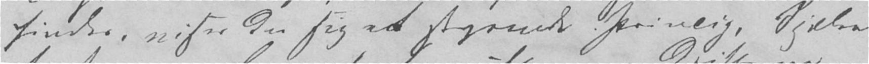findes, viser den sig et styrende Princip, Sjælen
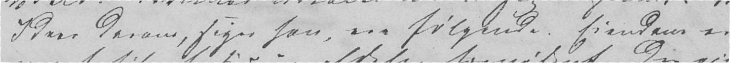Ideer derom, siger han, ere følgende. Eiendom er
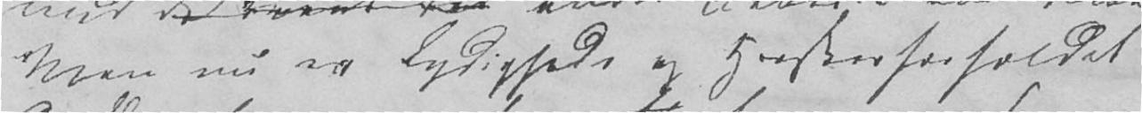Men nu er Lydigheds og Herskerforholdet
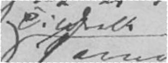Tildeels
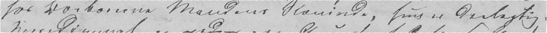hos Barbarerne Mandens Slavinde, hun er deelagtig i
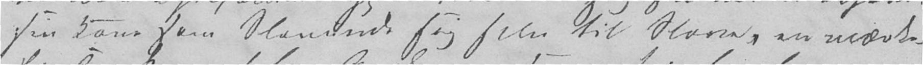sin kone som Slavinde sig selv til Slave, en mærke-
